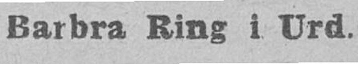Barbra Ring i Urd.
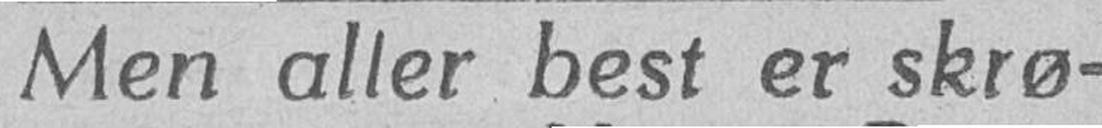Men aller best er skrø-
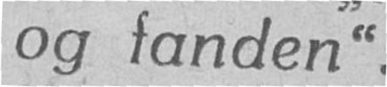og fanden".
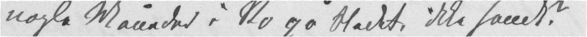nogle Måneder i Ro på Stedet, ikke sandt?
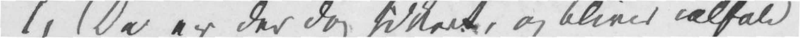1) De er der dog sikkert, og bliver ialfald
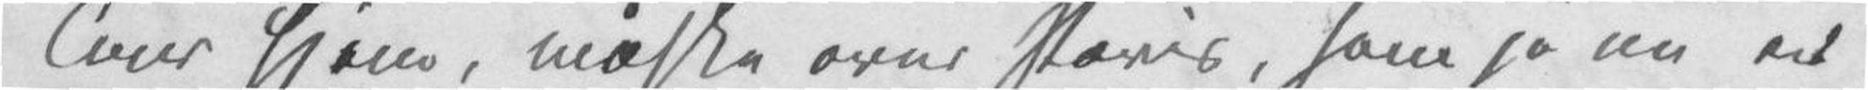Tour hjem, måske over Paris, som jo nu er
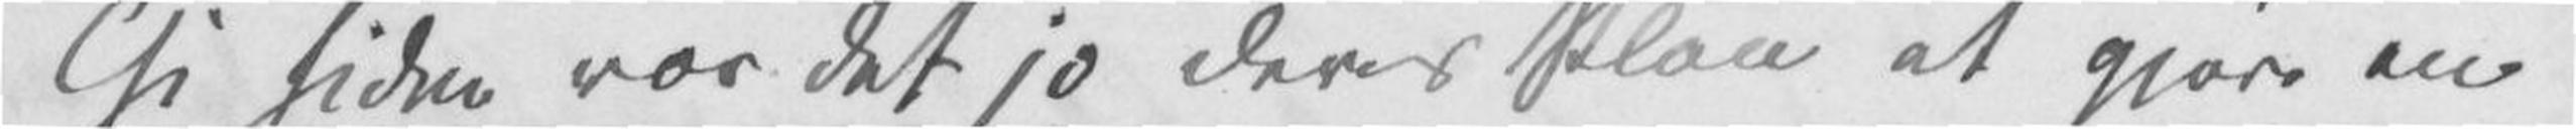Thi siden var det jo deres Plan at gjøre en
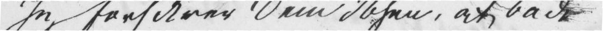Jeg forsikrer Dem Ibsen, at både
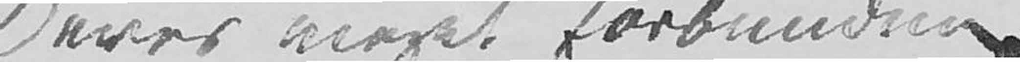Deres meget forbundne
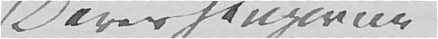Deres hengivne
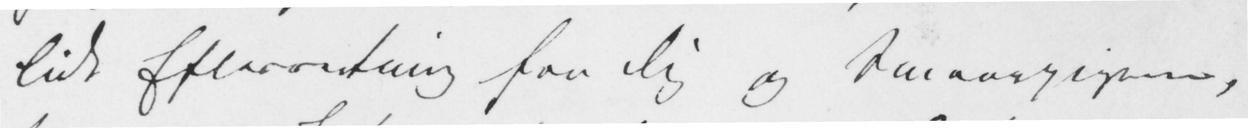lidt Efterretning fra Dig og Smaapigerne,
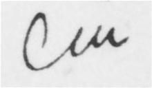Din
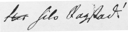<...> hils Rogstad!
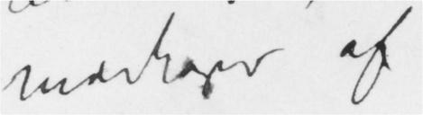modtages af
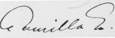Camilla C.
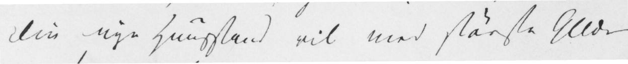Din nye Huustand vil med største Glæde
